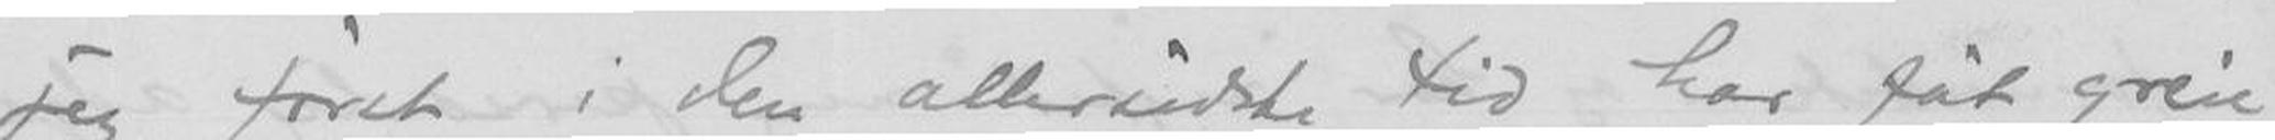jeg først i den allersidste tid har fåt greie
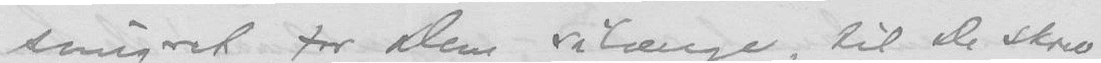smigret for Dem sålænge, til De skrev
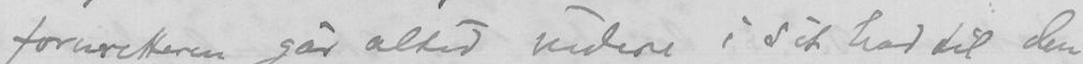foruretteren går altid videre i sit had til den
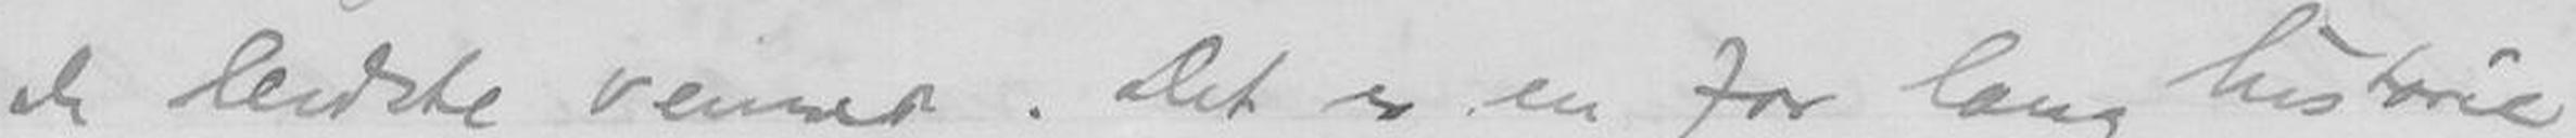de bedste venner. Det er en for lang historie
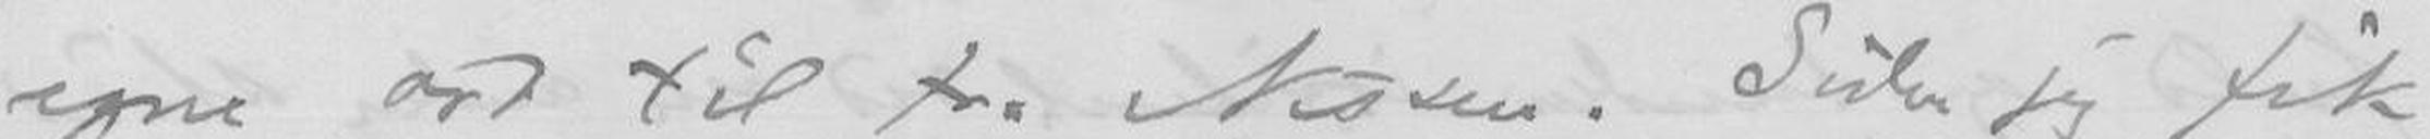egne ord til fr. Nissen. Siden jeg fik
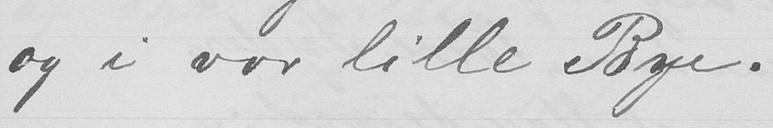og i vor lille Bye.
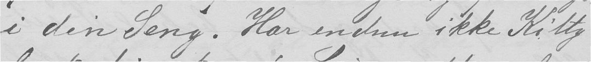i din Seng. Har endnu ikke Kitty
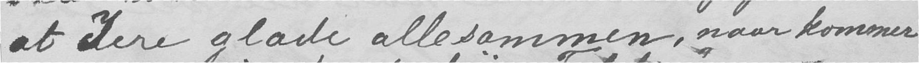at I ere glade alle sammen, naar kommer
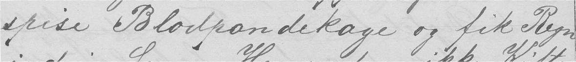spise Blodpandekage og fik Regn
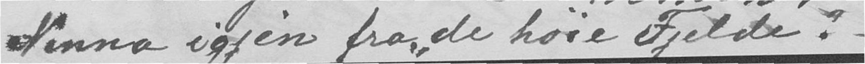Ninna igjen fra "de høie Fjelde." -
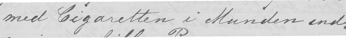med Cigaretten i Munden end-
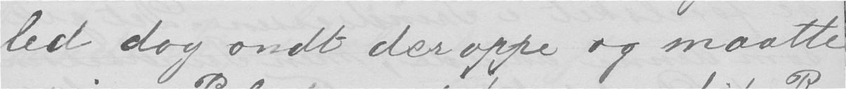led dog ondt deroppe og maatte
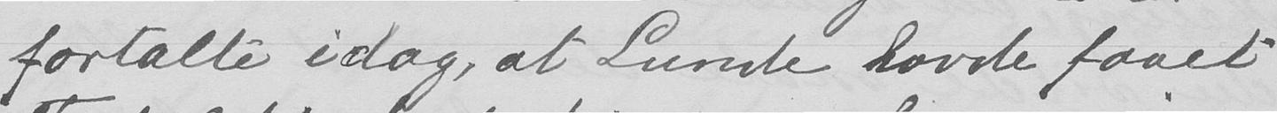fortalte idag, at Lunde havde faaet
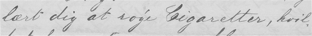lært dig at røge Cigaretter, hvil-
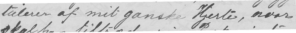tulerer af mit ganske Hjerte, naar
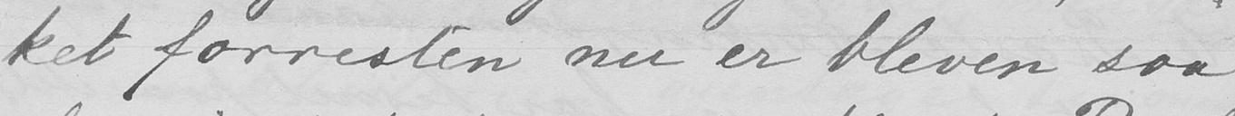ket forresten nu er bleven saa
